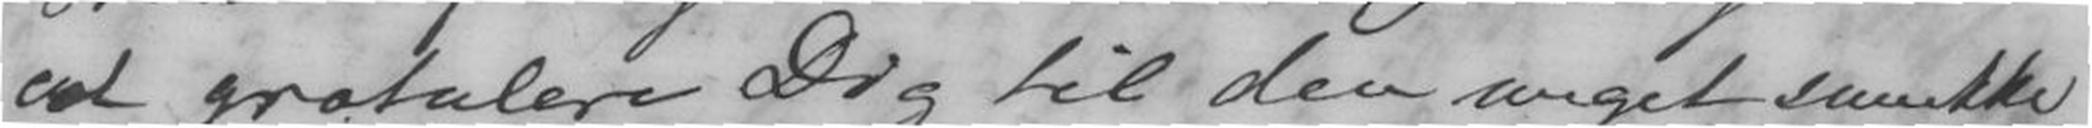at gratulere Dig til den meget smukke
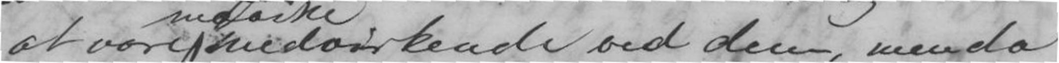at være medønkende ved dem, men da
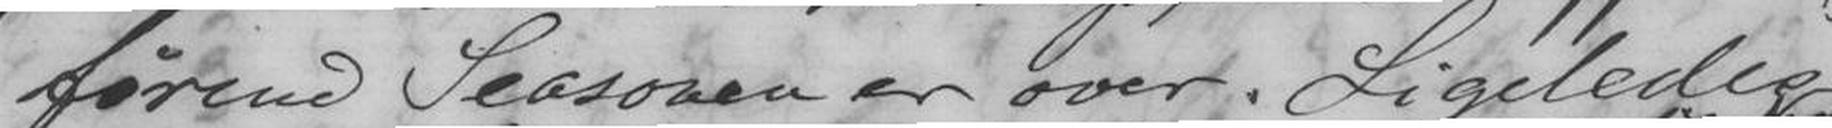førend Seasonen er over. Ligeledes
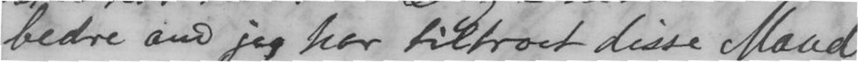bedre om jeg har tiltroet disse Mand
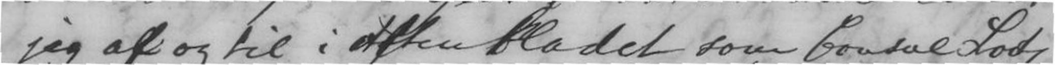jeg af og til i Aftenbladet som Consul Lotz
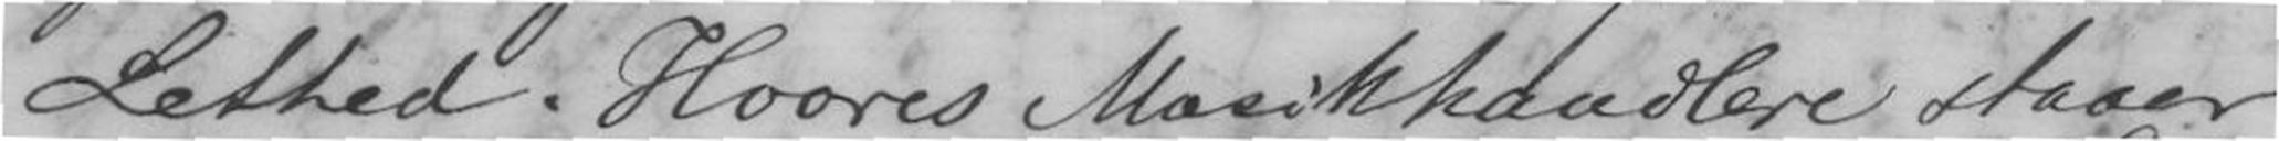Lethed. Hvores Musikhandlere staaer
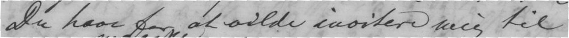Du have for at vilde inoitere mig til
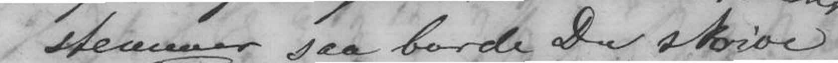Stemmer saa burde Du skrive
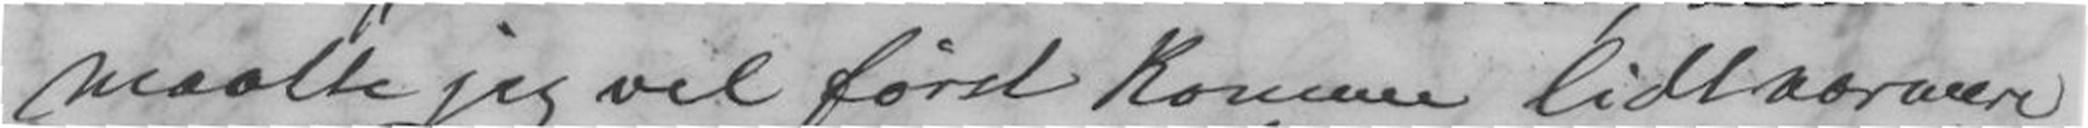maatte jeg vel først komme lidt nærmere
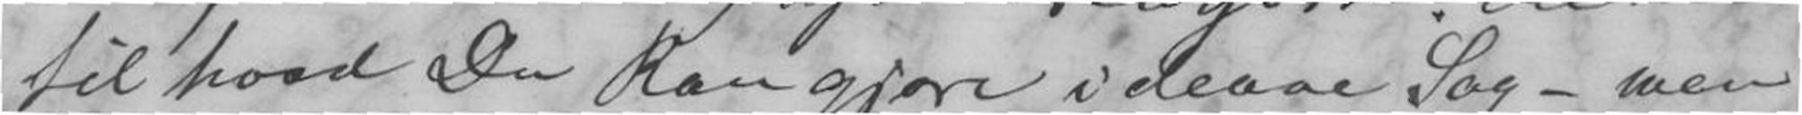til hvad Du kan gjøres i denne Sag - men
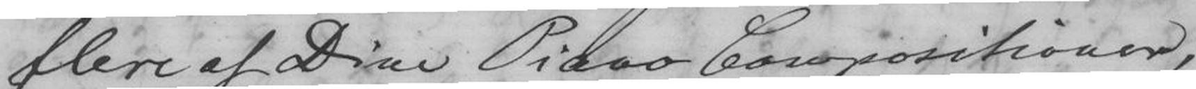flere af Dine Piano Conpositioner,
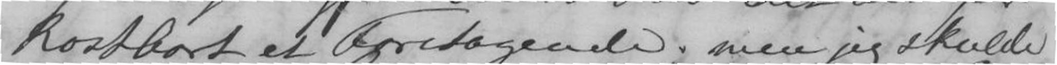kostbart et Foretagende; men jeg skulde
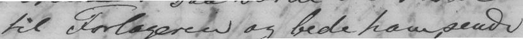til Forlægeren og bede ham sende
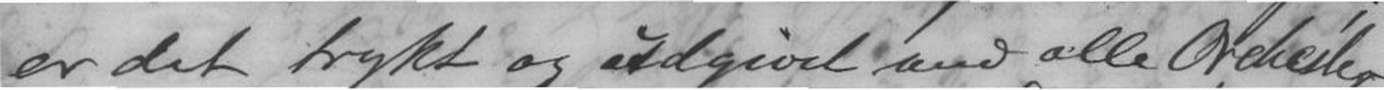er det trykt og udgivet med alle Orckester
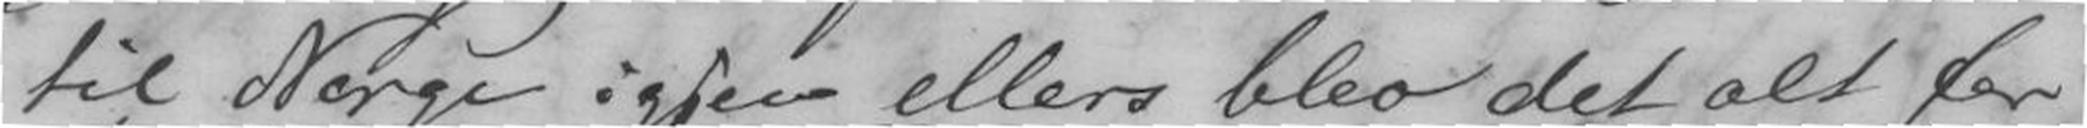til Norge igjen ellers blev det alt for
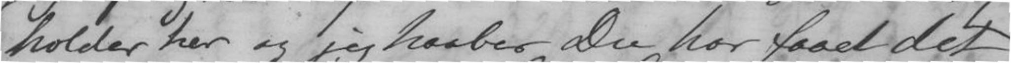holder her og jeg haaber Du har faaet det
